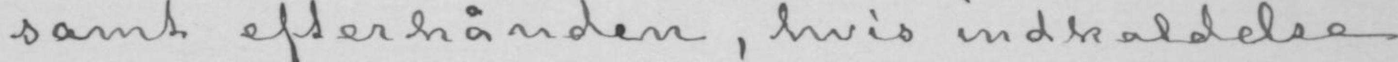samt efterhånden, hvis indkaldelse
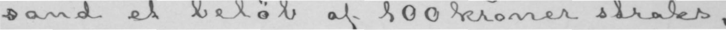sand et beløb af 100 kroner straks,
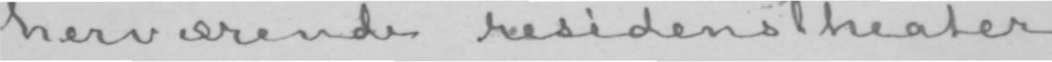herværende residenstheater.
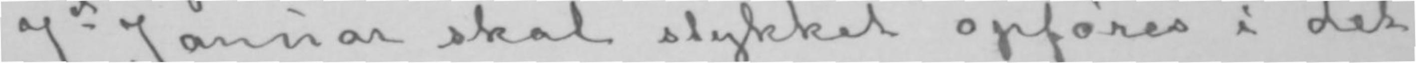7de Januar skal stykket opføres i det
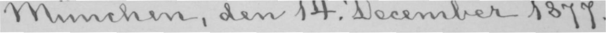München, den 14. December 1877.
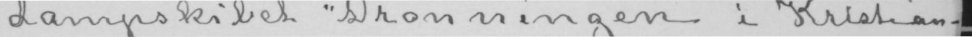dampskibet «Dronningen» i Kristian-
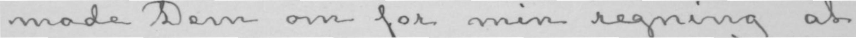mode Dem om for min regning at
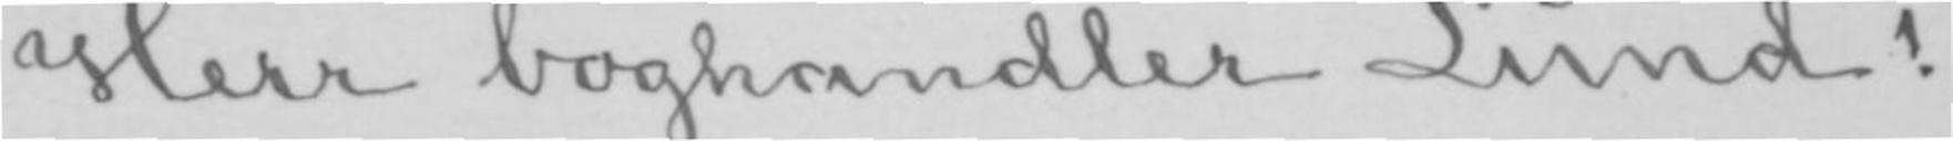Herr boghandler Lund!
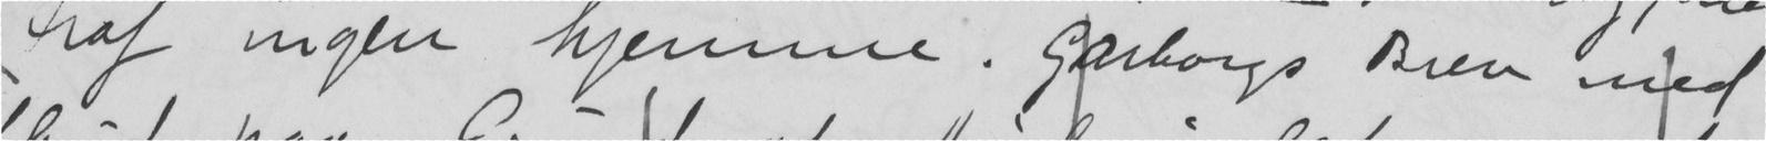traf ingen hjemme. Garborgs Brev med
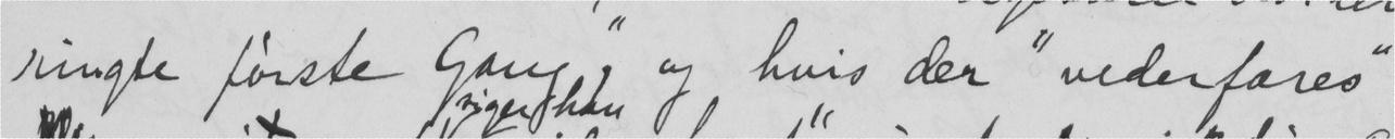ringte første Gang", og hvis der "vederfares"
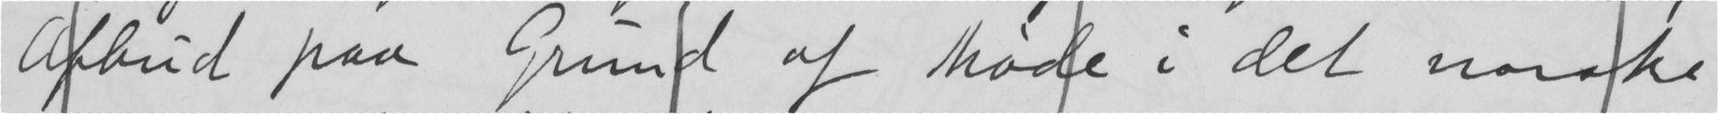Afbud paa Grund af Møde i det norske
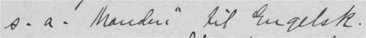s. a. Manden" til Engelsk.
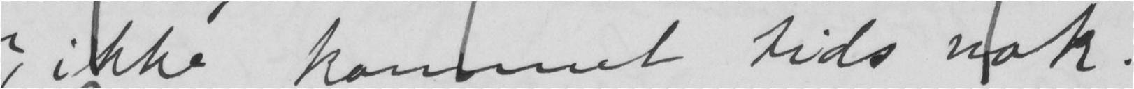ikke kommet tids nok.
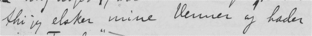thi jeg elsker mine Venner og hader
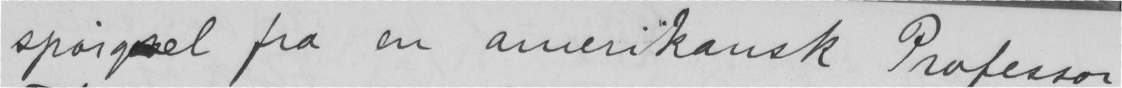spørgsel fra en amerikansk Professor
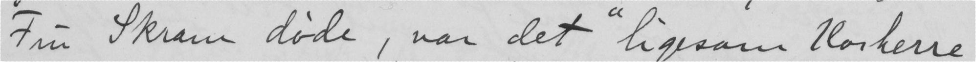Fru Skram døde, var det "ligesom Vorherre
